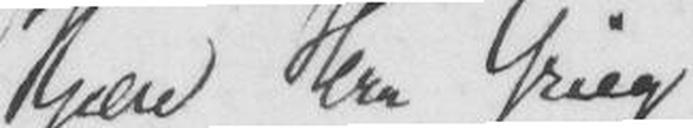Kjære Herr Grieg
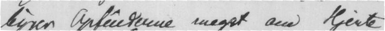meget om Hjerte
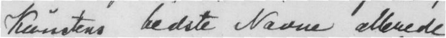Kunstens bedste Navne allerede
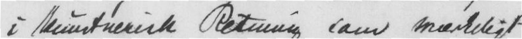i Kunstnerisk Retning som mærkeligt
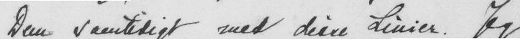Dem samtidigt med disse Linier. Jeg
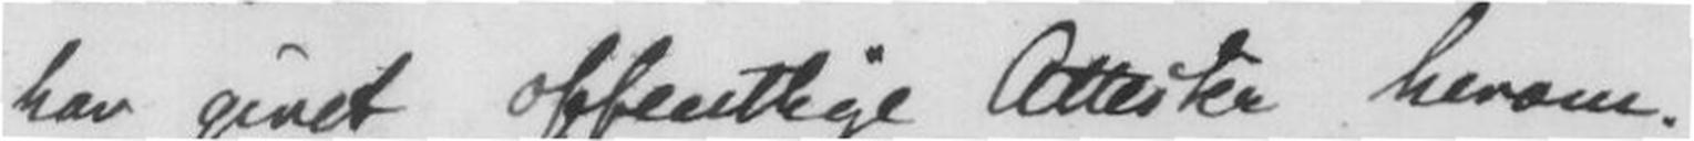har givet offentlige Attester herom.
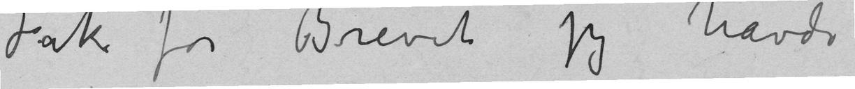Tak for Brevet Jeg havde
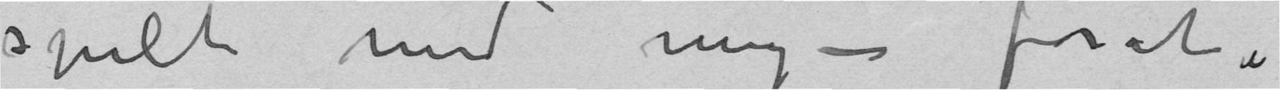spilt med mig – forat
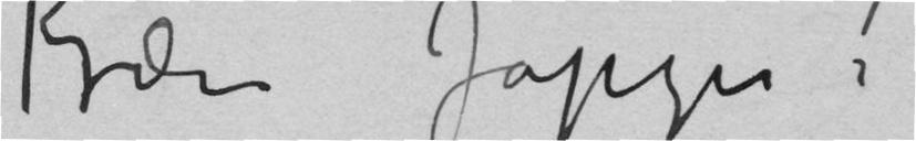Kjære Jappe!
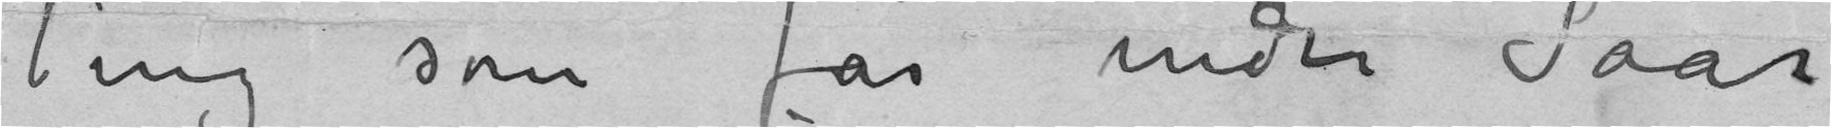Ting som får indre Saar
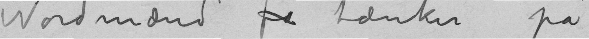Nordmænd f tænker på
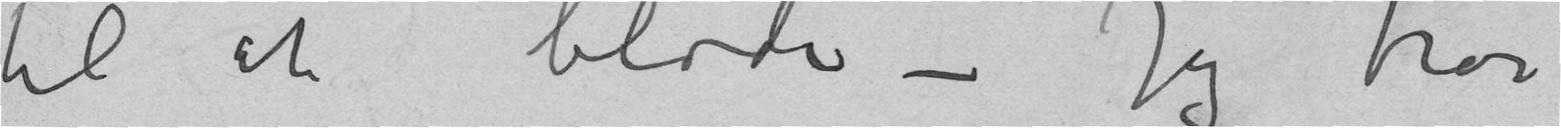til at bløde – Jeg tror
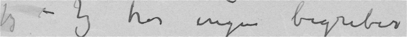jeg – Jeg har ingen begreber
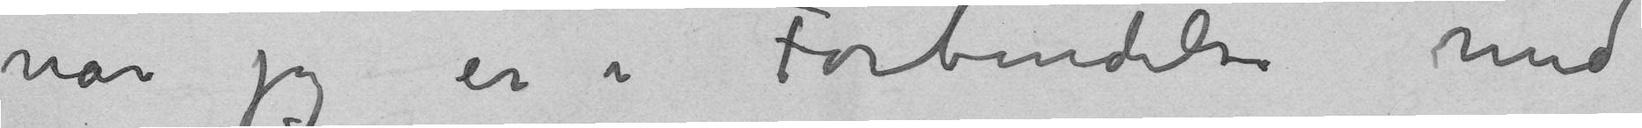når jeg er i Forbindelse med
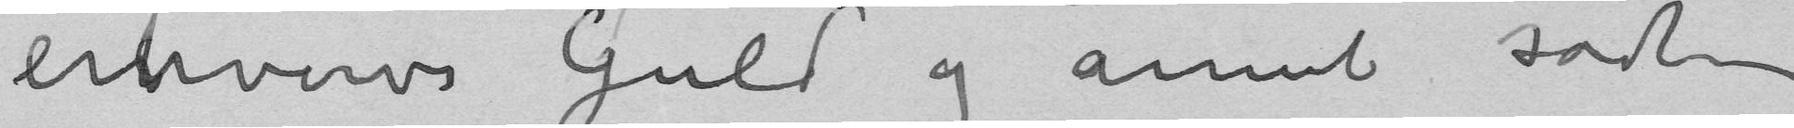erhverve Guld og annet sådan
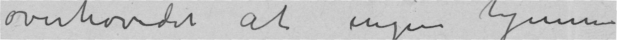overhodet at ingen hjemme
